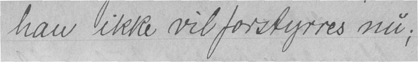han ikke vil forstyrres nu;
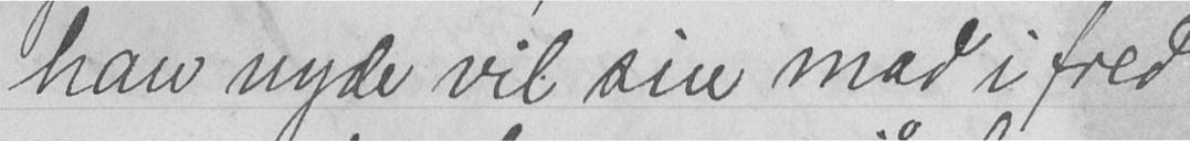han nyde vil sin mad i fred
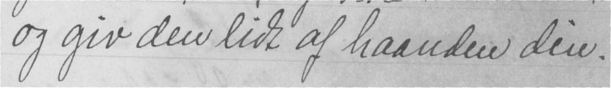og giv den lidt af haanden din.
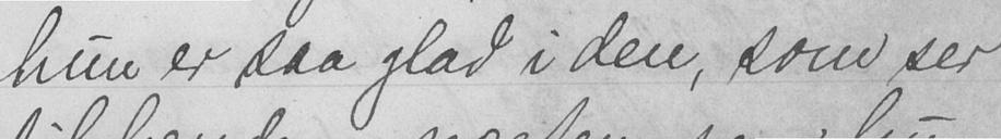hun er saa glad i den, som ser
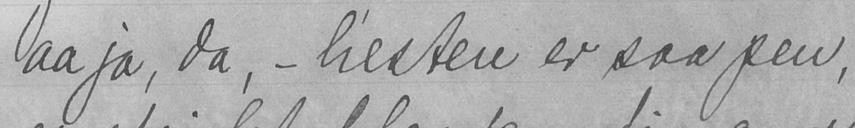aa ja, da, - hesten er saa pen,
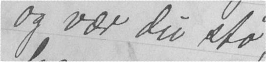og vær du stø,
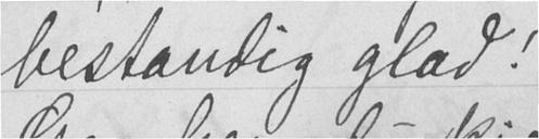bestandig glad!
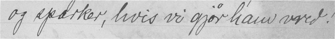og sparker, hvis vi gjør ham vred!
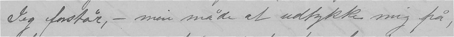Jeg forstår, - min måde at udtykke mig på,
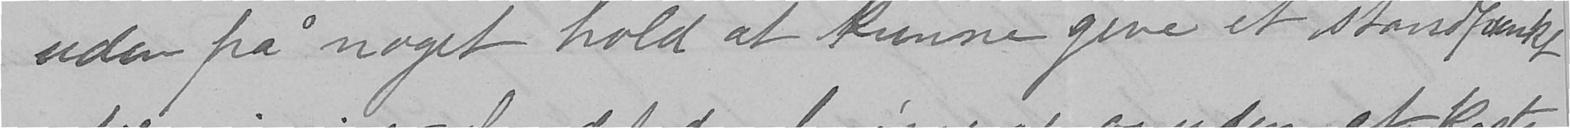uden på noget hold at kunne give et standpunkt
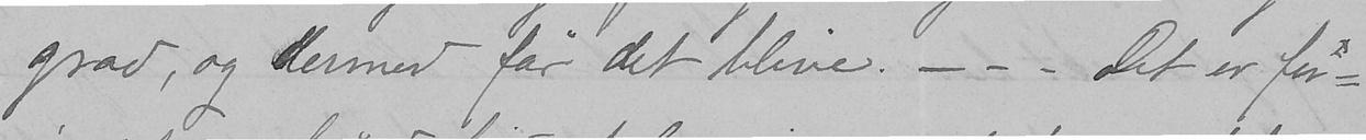grad, og dermed får det blive. - - - Det er for-
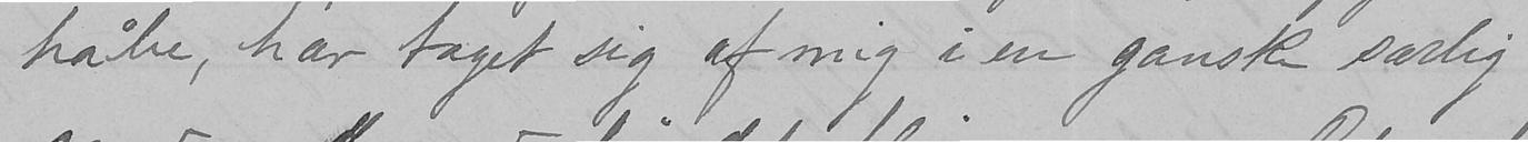håbe, har taget sig af mig i en ganske særlig
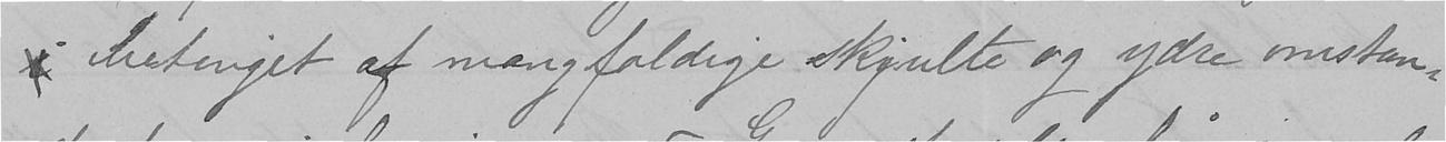i betinget af mangfoldige skjulte og ydre omstæn-
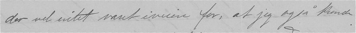der vel intet været iveien for, at jeg også kunde
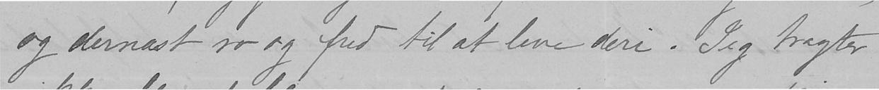og dernæst ro og fred til at leve deri. Jeg tragter
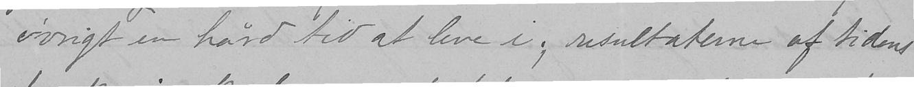øvrigt en hård tid at leve i, resultaterne af tidens
